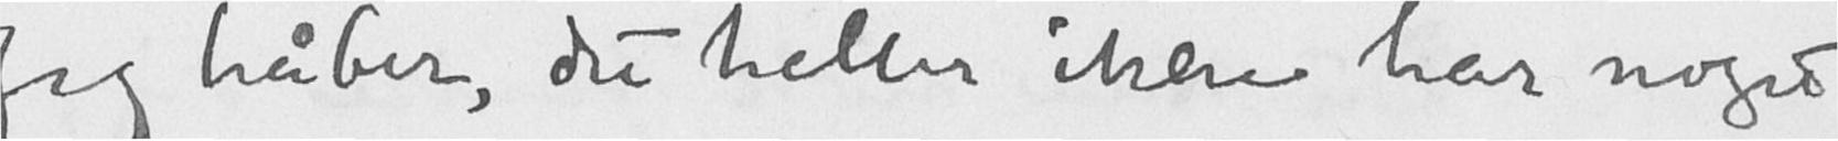Jeg håber, du heller ikke har noget
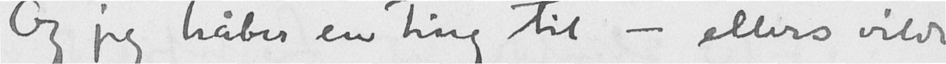Og jeg håber en ting til - ellers vilde
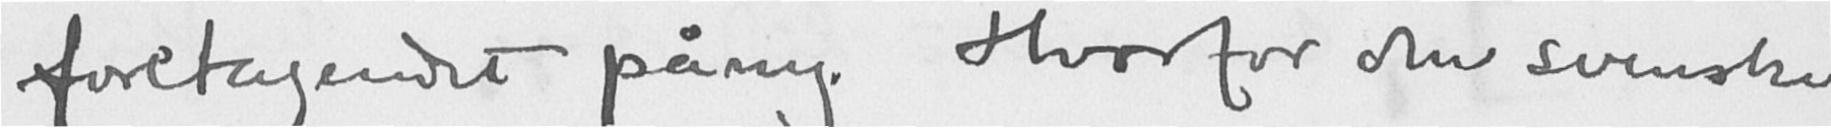foretagendet påny. Hvorfor den svenske
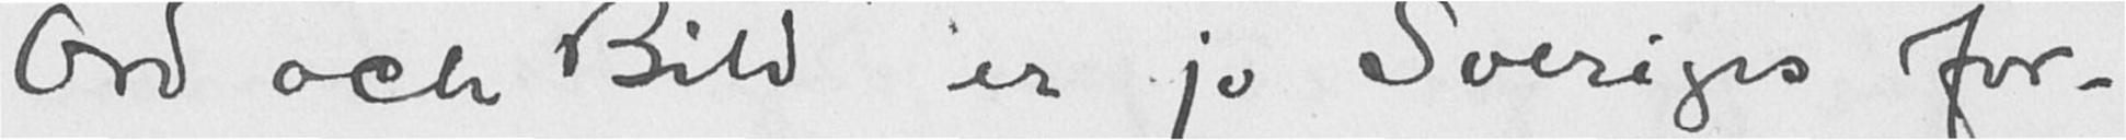"Ord och Bild" er jo Sveriges for-
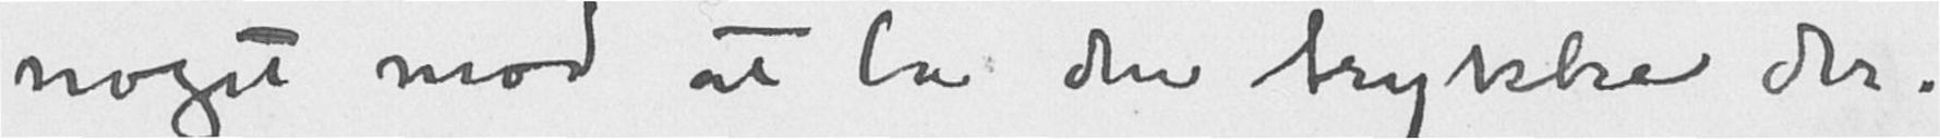noget mod at la den trykke der.
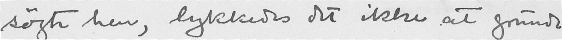søgte hen, lykkedes det ikke at grunde
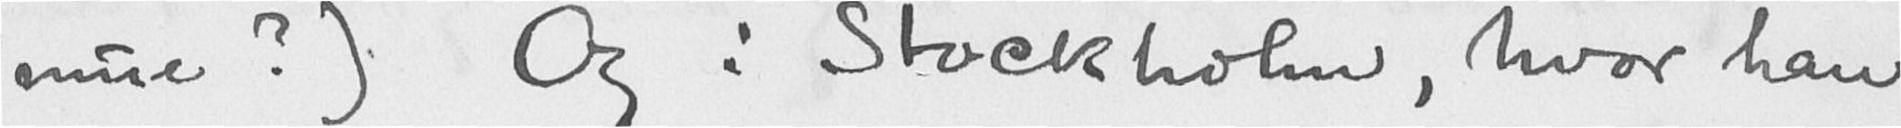mue?)  Og i Stockholm, hvor han
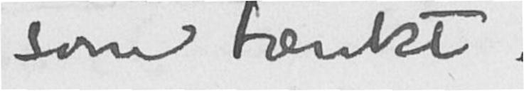som tænkt.
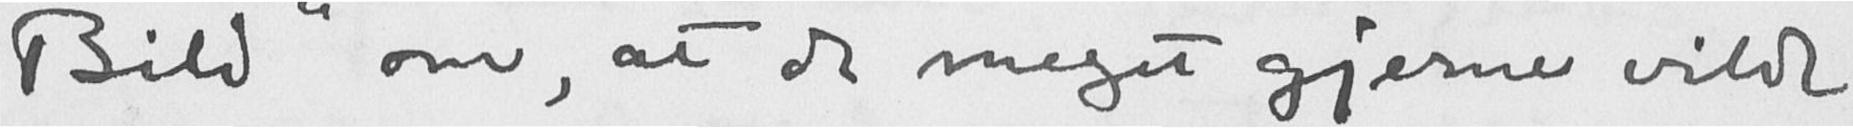Bild" om, at de meget gjerne vilde
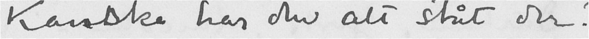Kanske har du alt ståt der?
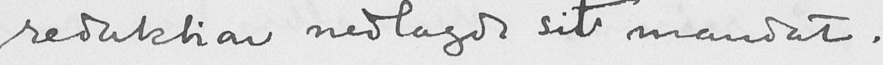redaktion nedlagde sit mandat.
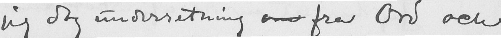jeg dog underretning fra "Ord och
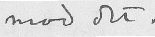mod det.
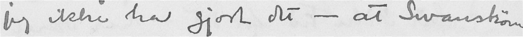jeg ikke har gjort det - at Swanstrøm
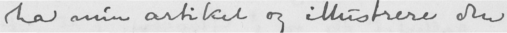ha min artikel og illustrere den
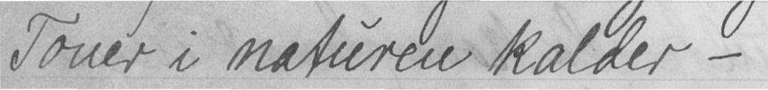Toner i naturen kalder -
